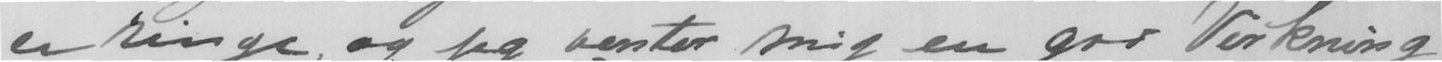er ringe, og jeg venter mig en god Virkning
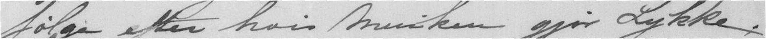følge etter hvis Musiken gjør Lykke;
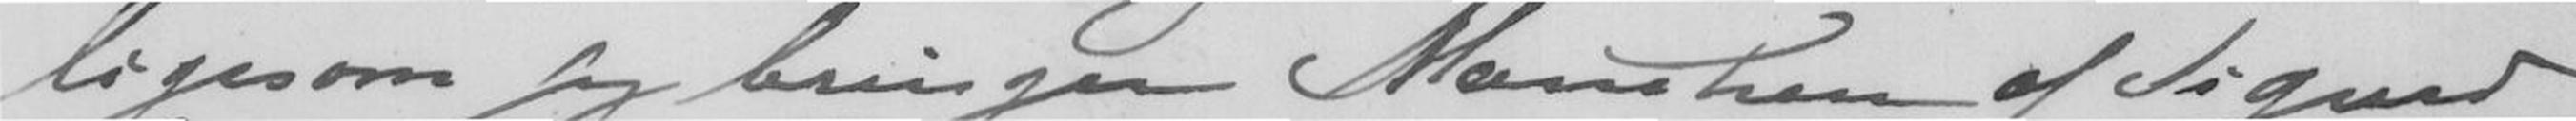ligesom jeg bringer Marschen af Sigurd
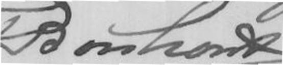F. Borchorst.
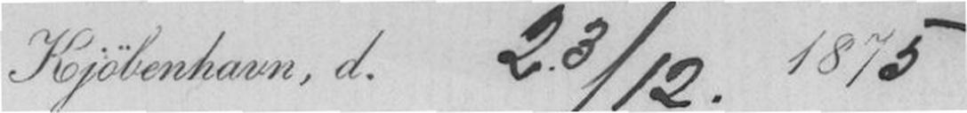Kjøbenhavn, d. 23/12 1875
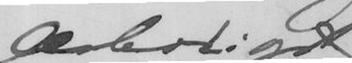Ærbødigst
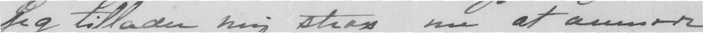jeg tillader mig strax nu at anmode
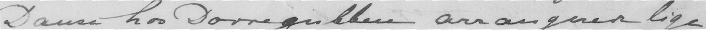Danse hos Dovregubben arrangerede lige-
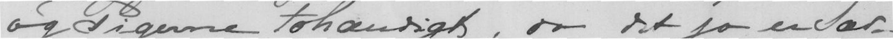og Pigerne Tohændigts, da det jo er Sæd-
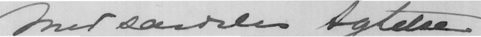Med særdeles Agtelse
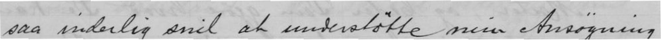saa inderlig snil at understøtte min Ansøgning
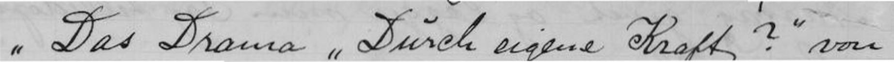"Das Drama "Dürch eigene Kraft?" von
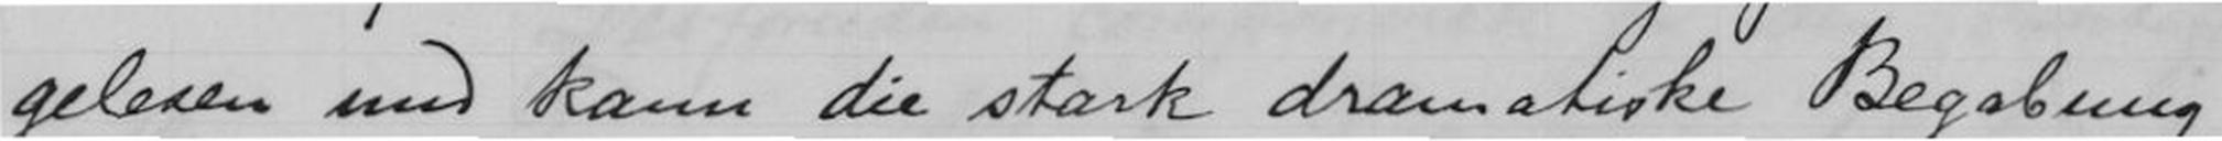gelesen und kann die stark dramatiske Begsbung
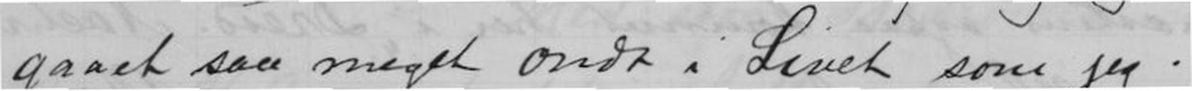gaaet saa meget ondt i livet som jeg.
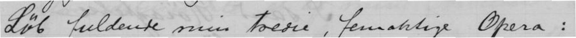Løb fuldende min Tredie, femaktige Opera:
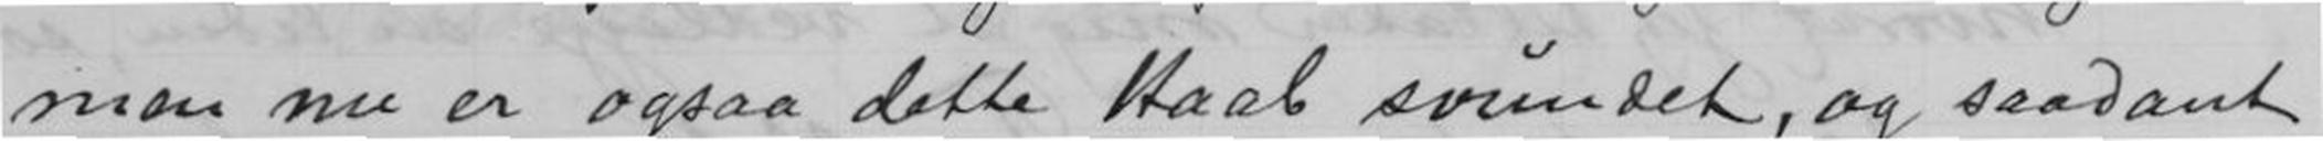man nu er ogsaa dette Haab svundet, og saadant
